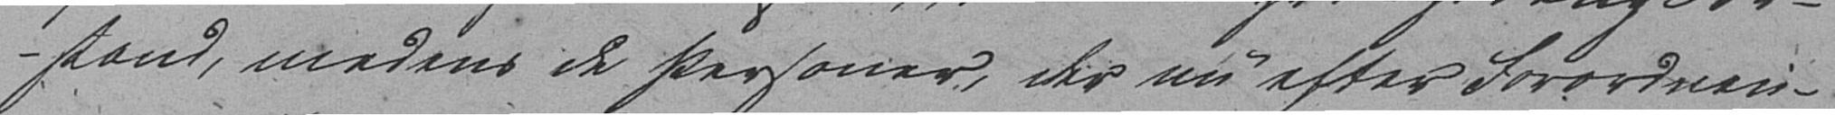stand, medens de Personer, der nu efter Forordnin-
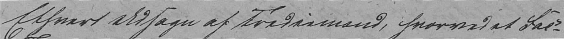Ethvert Udsagn af Trediemand, hvorved et Fac-
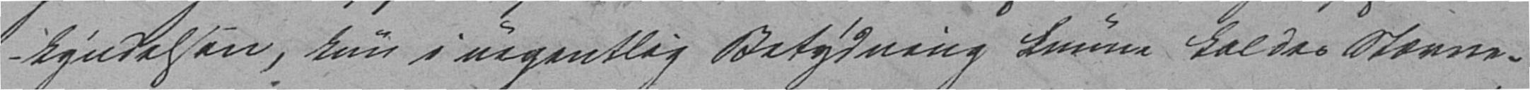kyndelsen, kun i uegentlig Betydning kunne kaldes Stævne-
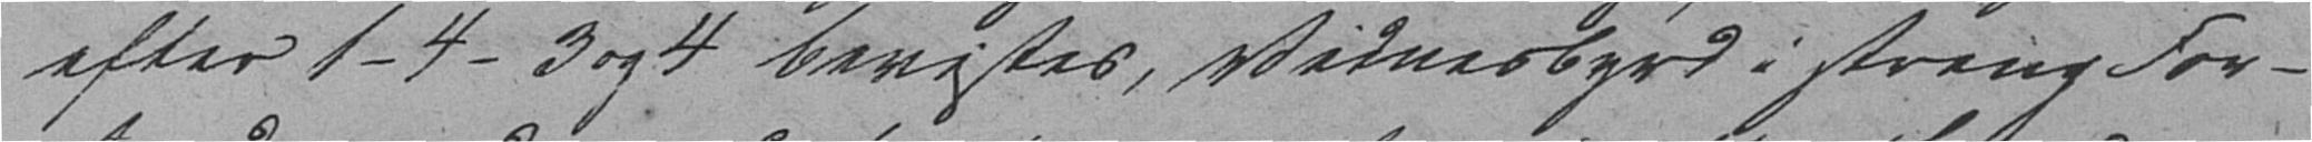efter 1-4-3 og 4 bevistes, Vidnesbyrd i streng For-
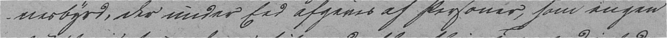nesbyrd, der under Eed afgives af Personer, som ingen
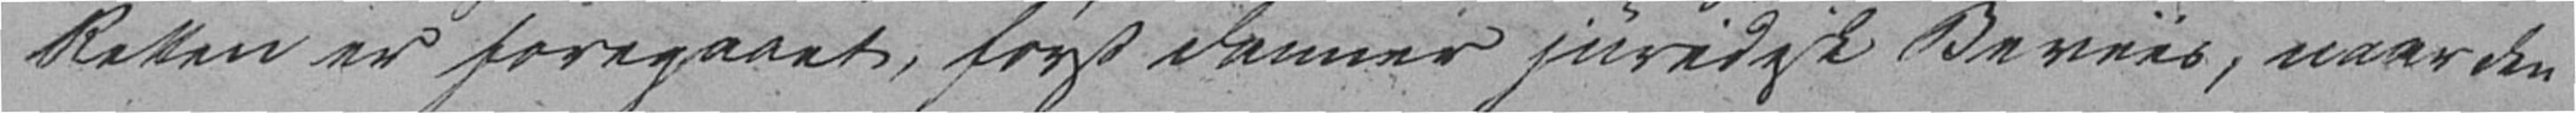Retten er foregaaet, først danner juridisk Beviis, naar den
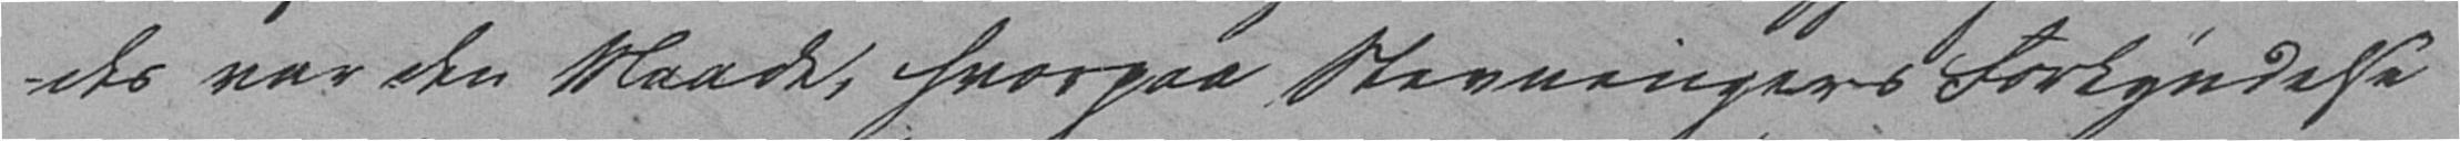des var den Maade, hvorpaa Stevningers Forkyndelse
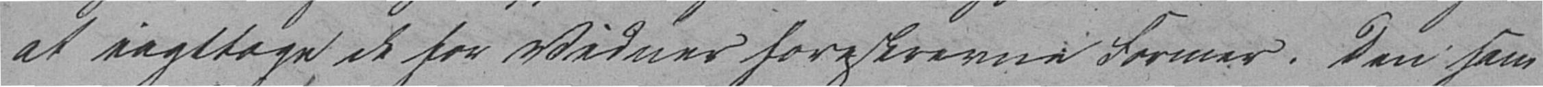at iagttage de fra Vidner foreskrevne Former. Den sam-
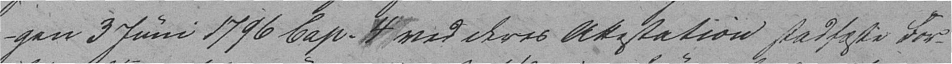gen 3 Juni 1796 Cap. 4 ved deres Attestation stadfæste For-
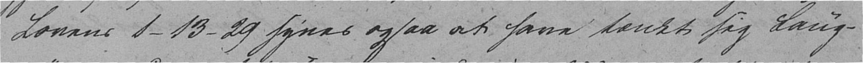Lovens 1-13-29 synes ogsaa at have tænkt sig Laug-
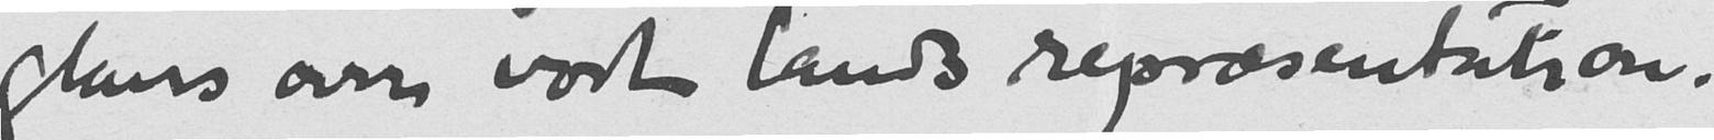glans over vort lands repræsentation.
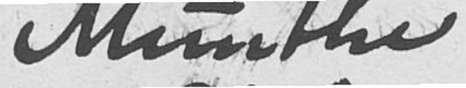Munthe
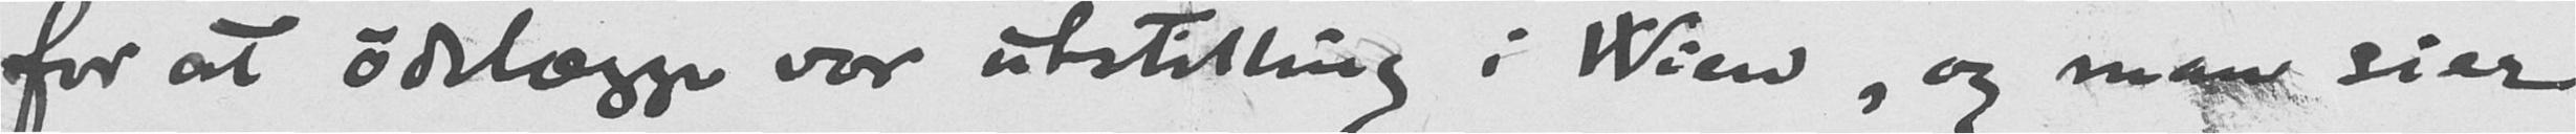for at ødelægge vor utstilling i Wien, og man sier
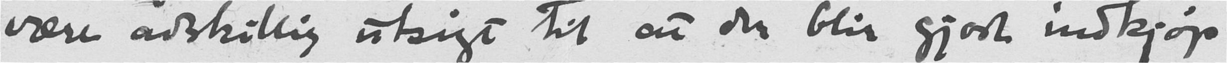være adskillig utsigt til at der blir gjort indkjøp
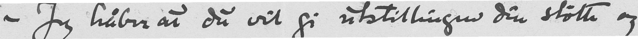- Jeg håber at du vil gi utstillingen din støtte og
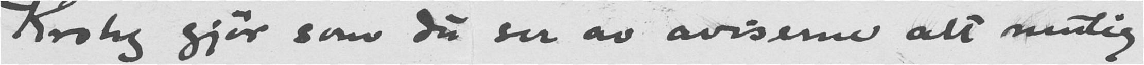Krohg gjør som du ser av avisene alt mulig
